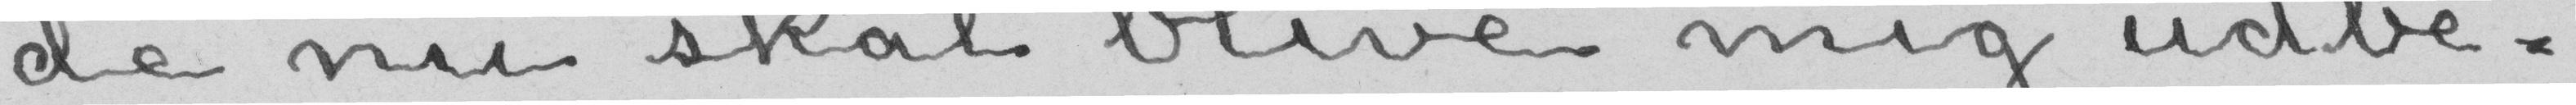de nu skal blive mig udbe-
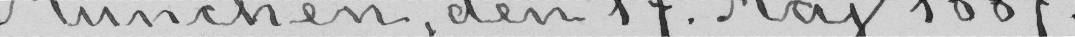München, den 17. Maj 1887.
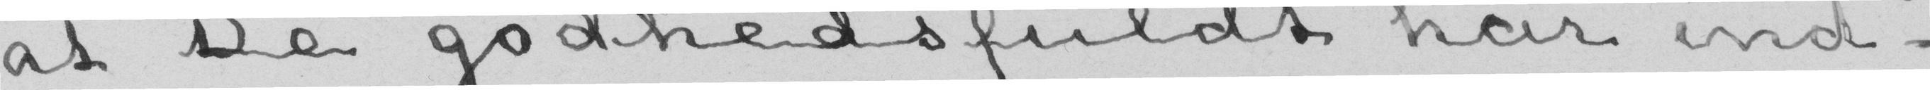at De godhedsfuldt har ind-
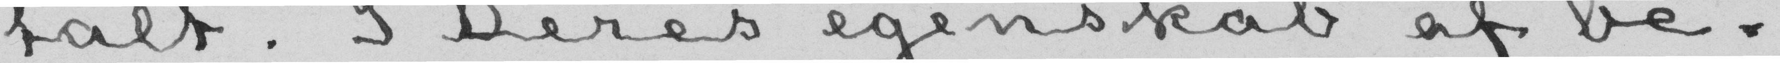talt. I Deres egenskab af be-
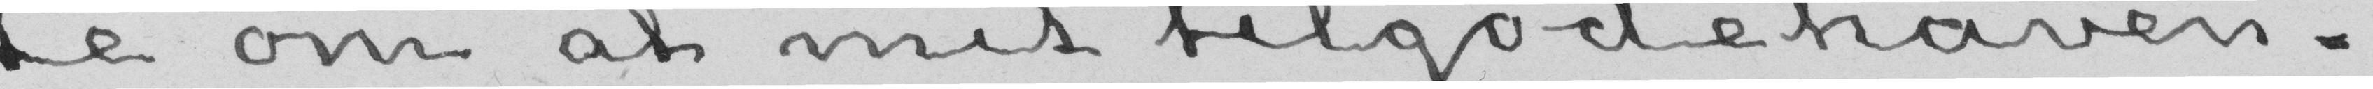te om at mit tilgodehaven-
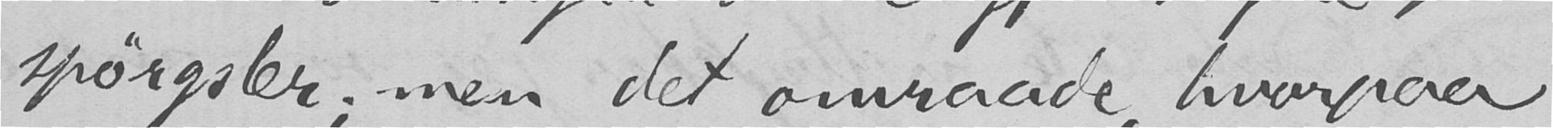spørgsler; men det omraade, hvorpaa
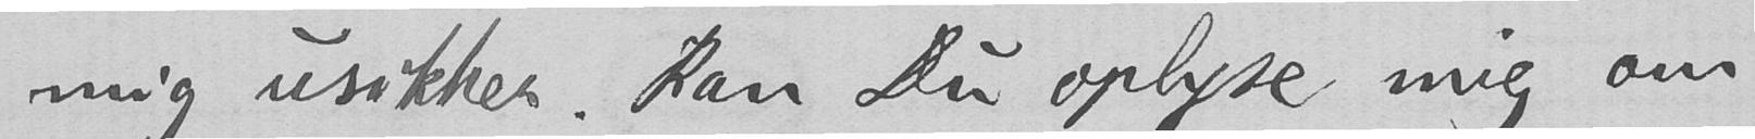mig usikker. Kan Du oplyse mig om
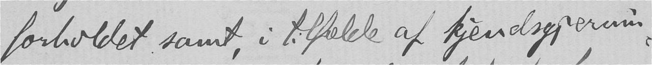forholdet samt, i tilfælde af kjendsgjernin-
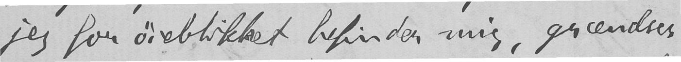jeg for øieblikket befinder mig, grændser
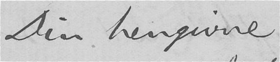Din hengivne
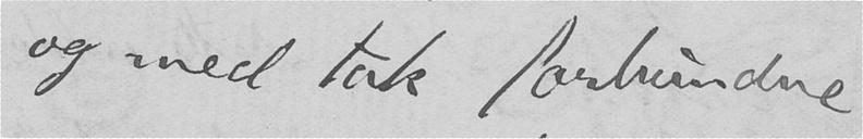og med tak forbundne
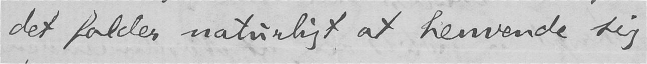det falder naturligt at henvende sig
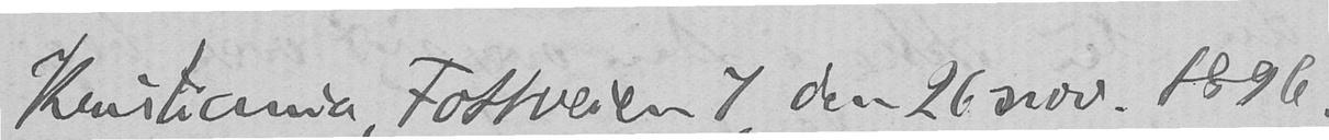Kristiania, Fossveien 7, den 26 nov. 1896.
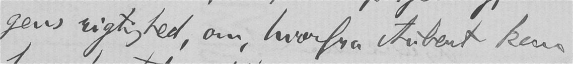gens rigtighed, om, hvorfra Aubert kan
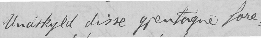Undskyld disse gjentagne fore-
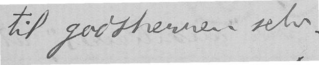til godsherren selv.
